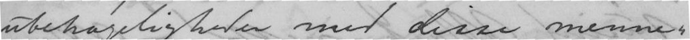ubehageligheder med disse menne-
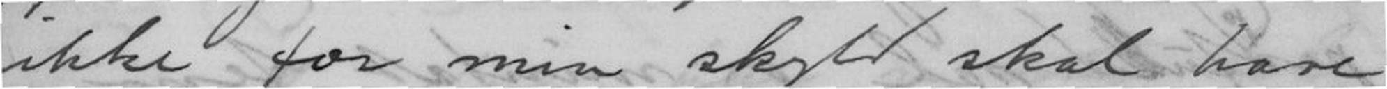ikke for min skyld skal have
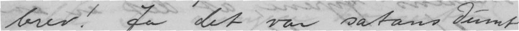brev! Ja det var satans dumt
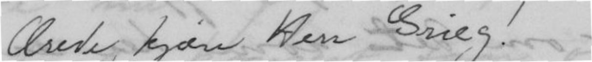Ærede, kjære Herr Grieg!
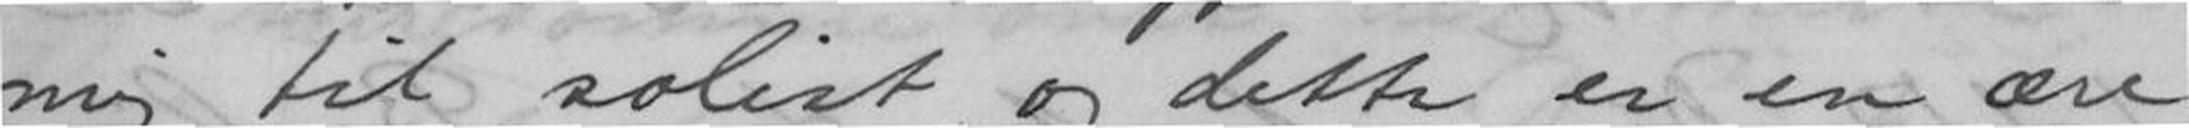mig til solist, og dette er en ære
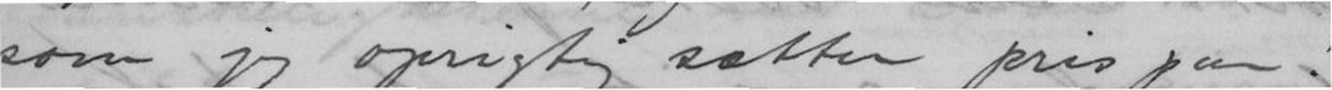som jeg oprigtig sætter pris paa!
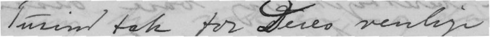Tusind tak for Deres venlige
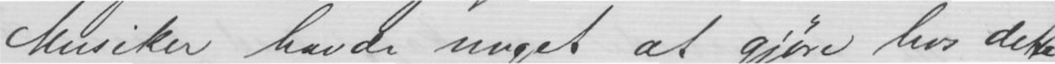Musiker havde noget at gjøre hos dette
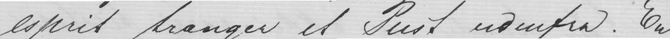esprit trænger et Pust udenfra. En
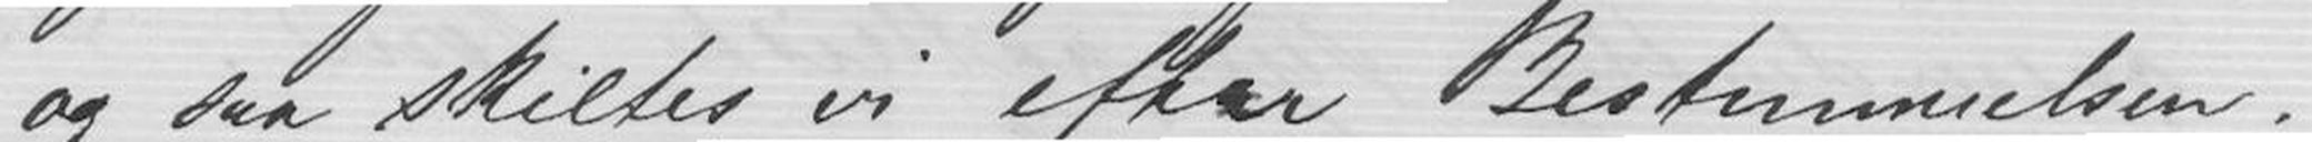og saa skiltes vi efter Bestemmelsen.
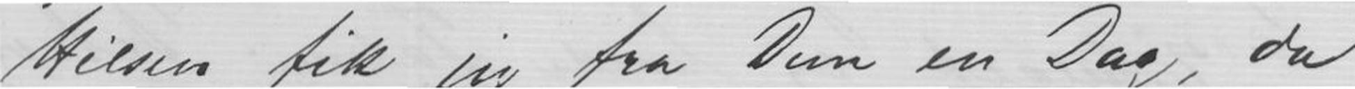Hilsen fik jeg fra Dem en Dag, da
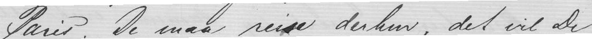Paris. De maa rise derhen, det vil De
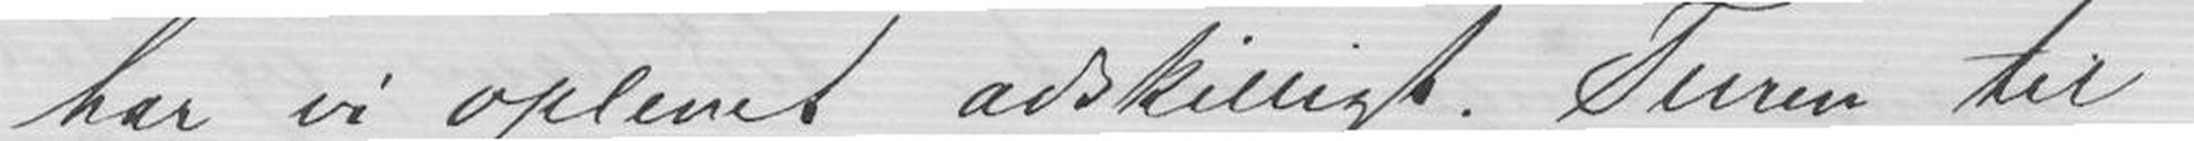har vi oplevet adskilligt. Turen til
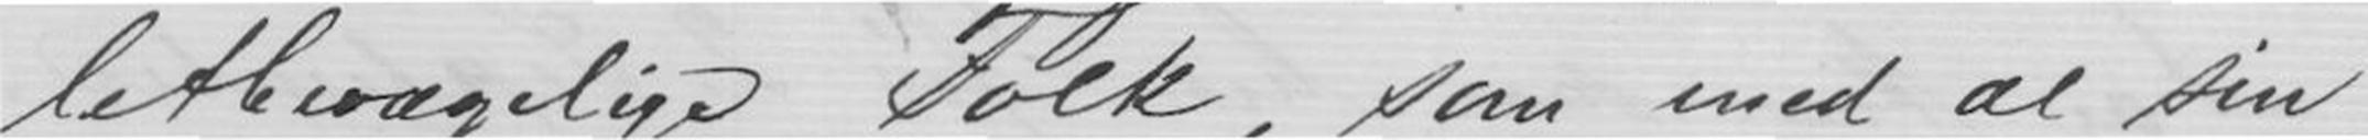letbevægelige Folk, som med al sin
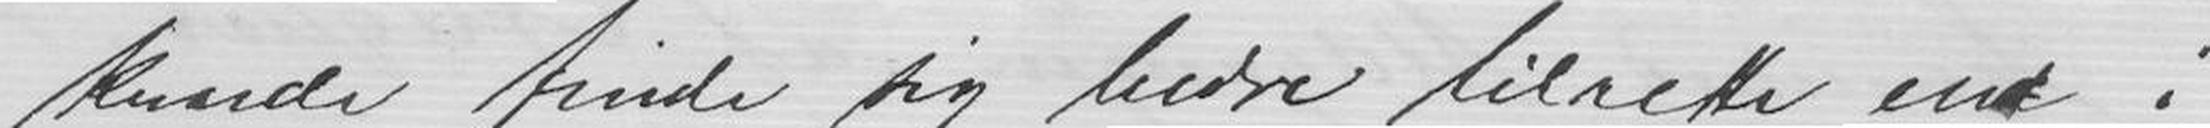kunde finde sig bedre tilrette end i
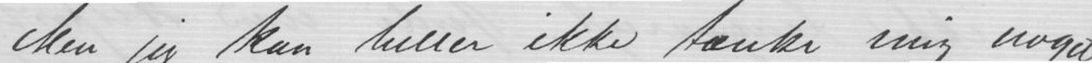Men jeg kan heller ikke tænke mig noget
